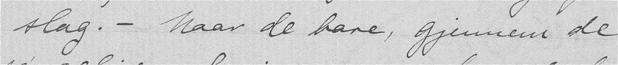slag. - Naar de bare, gjennem de
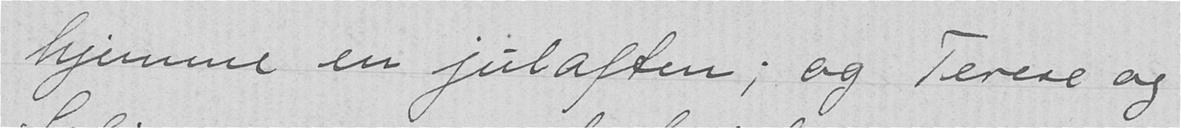hjemme en julaften; og Terese og
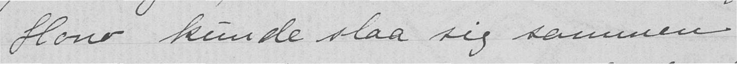Hono kunde slaa sig sammen
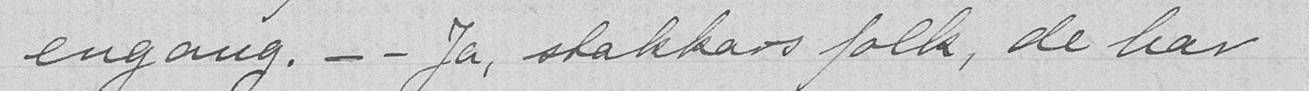engang. - - Ja, stakkars folk, de har
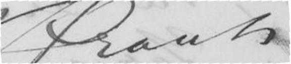Frants
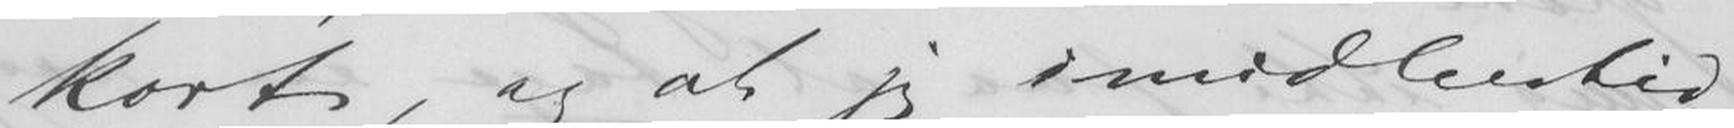kort, og at jeg imidlertid
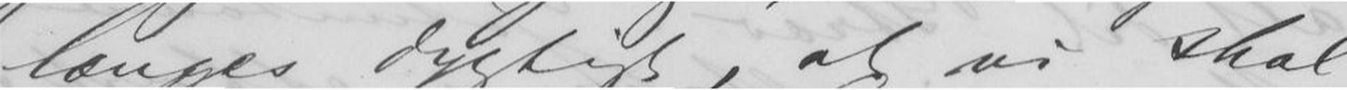længes dygtigt, at vi skal
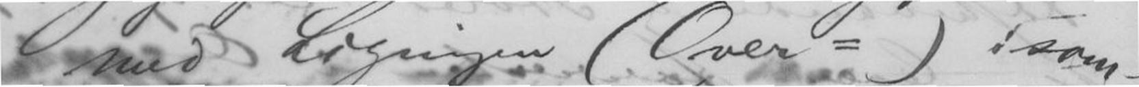med Ligningen (Over =) i som-
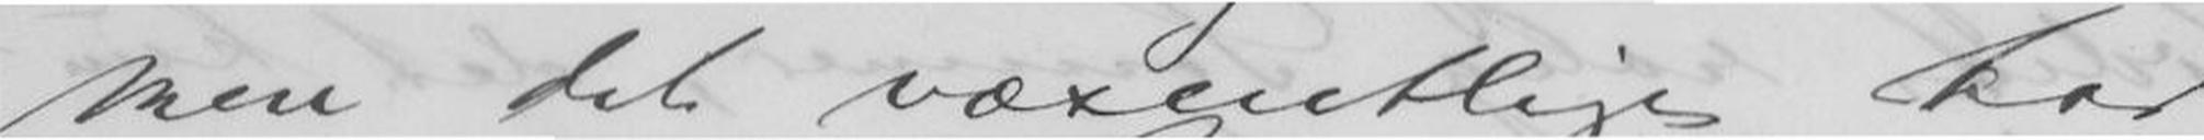men det væsentlige har
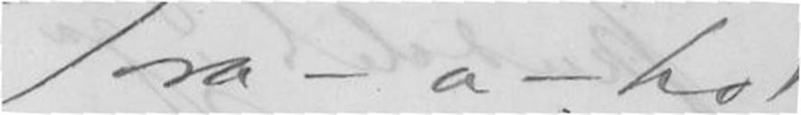Tra - a - ho!
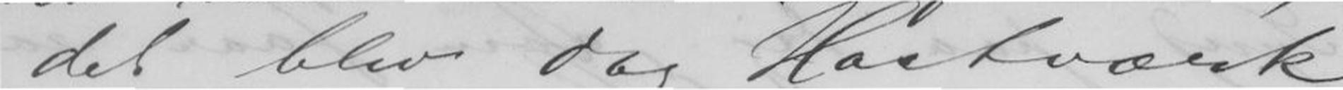- det blev dog Hastværk,
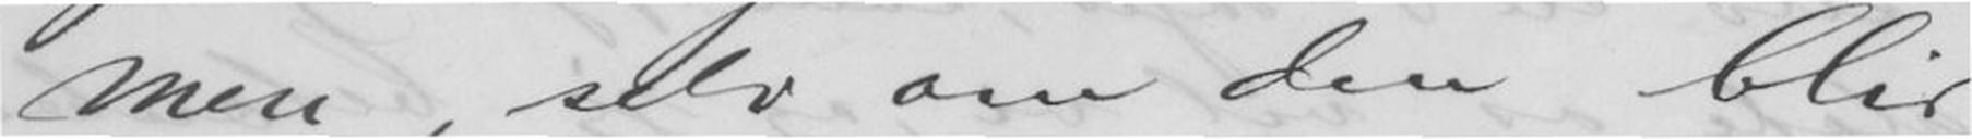men, selv om den blir
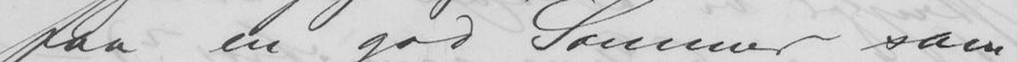faa en god Sommer sam-
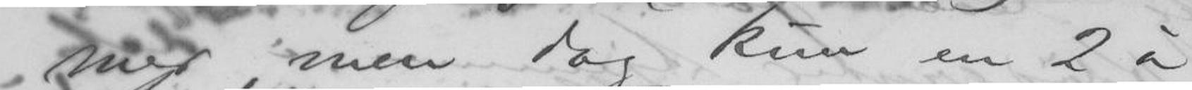mer, men dog kun en 2 à
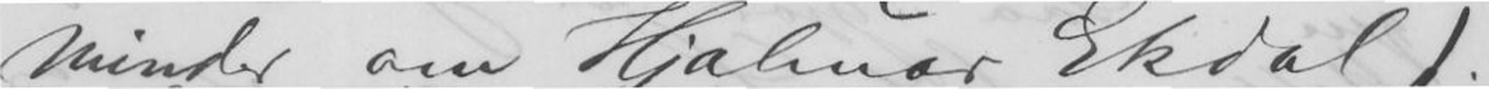minder om Hjalmer Ekdal).
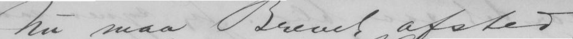Nu maa Brevet afsted,
